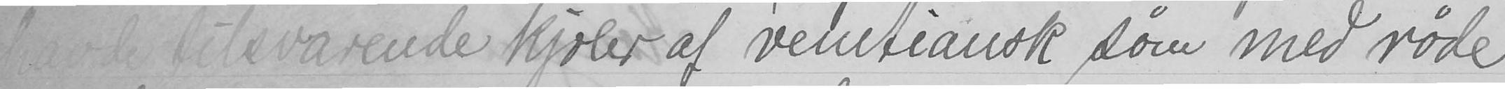havde tilsvarende kjoler af venetiansk søm med røde
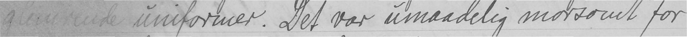gimirende uniformer. Det var umaadelig morsomt for
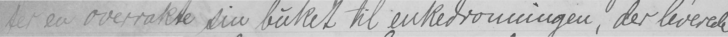ter en overrrakte sin buket til enkedronningen, der leverede
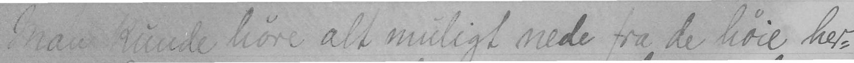Man kunde høre alt muligt nede fra de høie her-
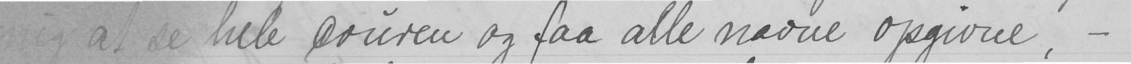mig at se hele couren og faa alle navne opgivne, -
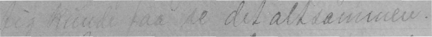lig kunde faa se det alt sammen.
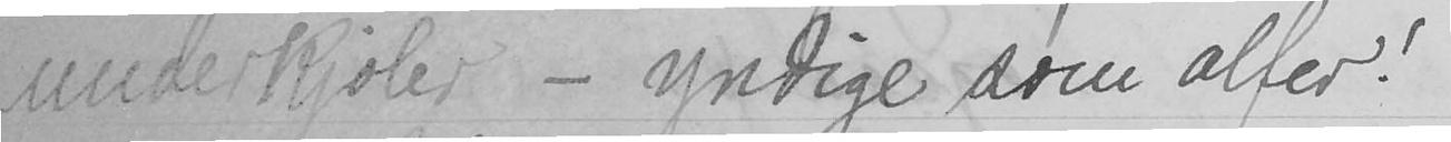underkjoler - yndige som alfer!
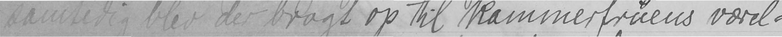samtidig blev der bragt op til kammerfruens værel-
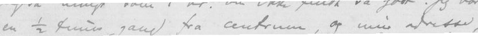en ½ times gang fra centrum, og min adresse
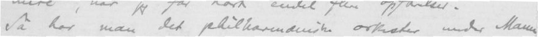Så har man det philharmonisk orkester under Mann-
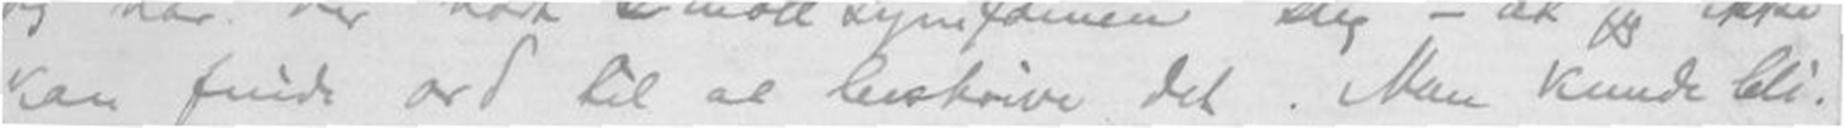kan finde ord til at beskrive det. Man kunde bli-
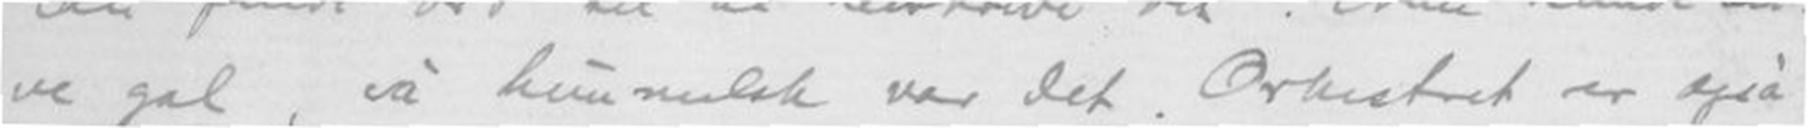ve gal, så himmelsk var det. Orkestret er også
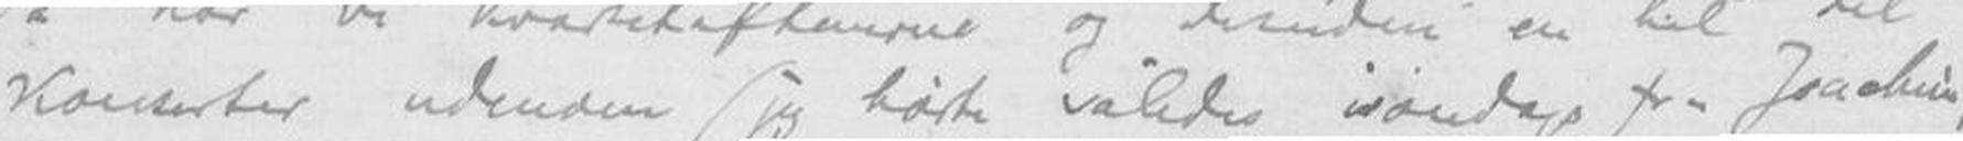konserter udenom (jeg hørte således isøndags fr. Joachim,
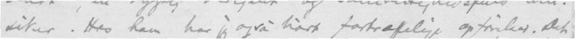siker. Hos ham har jeg også hørt fortræffelige opførelser. Det
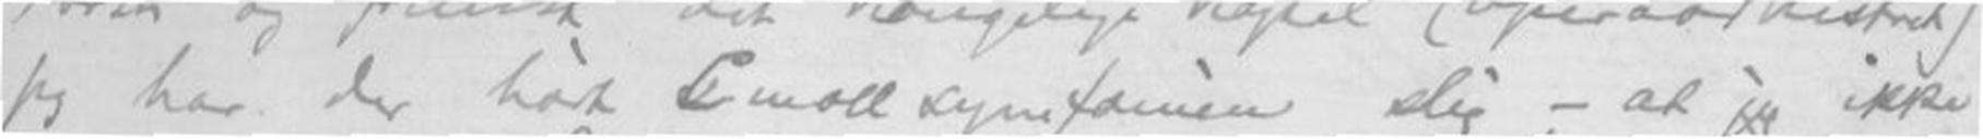Jeg har der hørt C moll symfonien slig - at jeg ikke
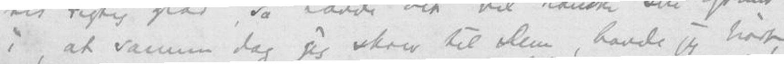i, at samme dag jeg skrev Dem, havde jeg hørt,
